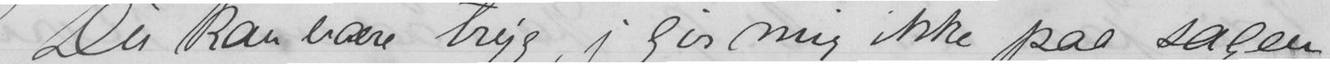Du kan være tryg, jeg gir mig ikke paa sagen
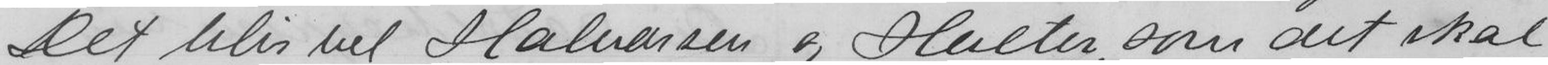Det blir vel Halvorsen og Holter som det skal
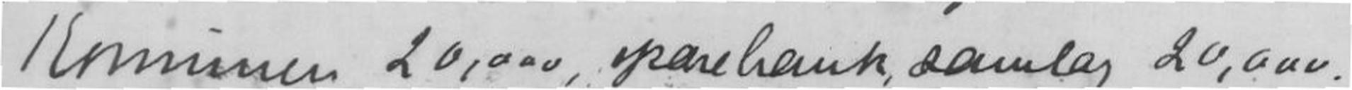Komunen 20,000, sparebank, samlag 20,000!
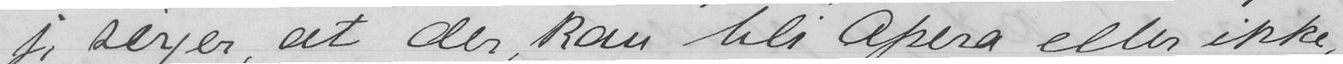jeg siger, at der kan bli Opera eller ikke,
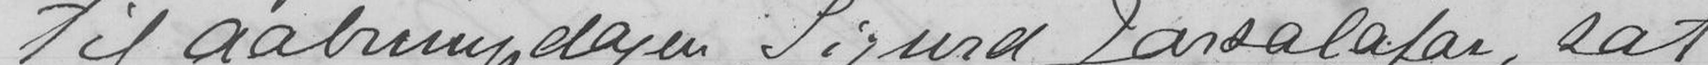Til Aabningsdagen Sigurd Jorsalfar, sat
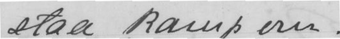staa kamp om!
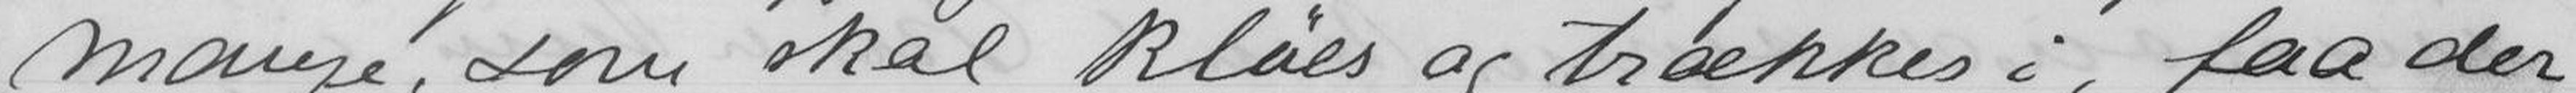mange, som skal kløes og trækkes i, faa der
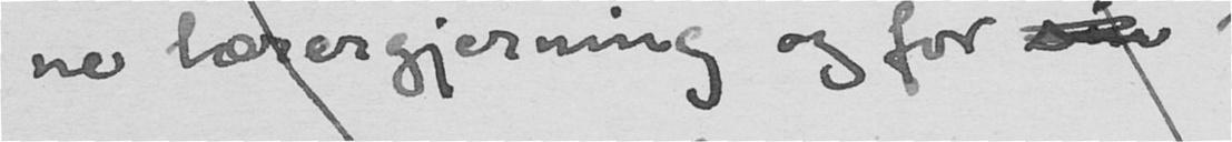ne lærergjerning og for sin
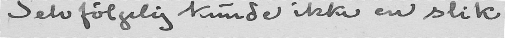Selvfølgelig kunde ikke en slik
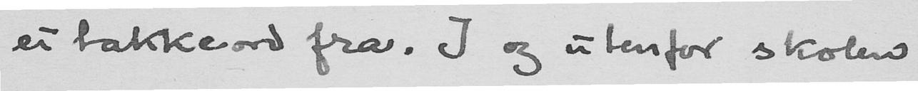et takkeord fra. I og utenfor skolen
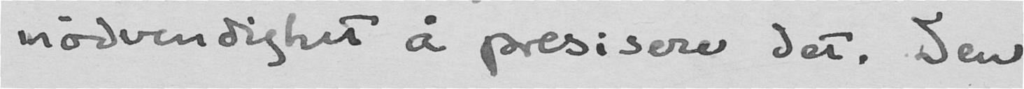nødvendighet å presisere det. Den
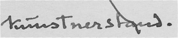kunstnerstand
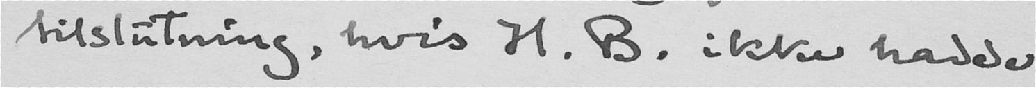tilslutning, hvis H. B. ikke hadde
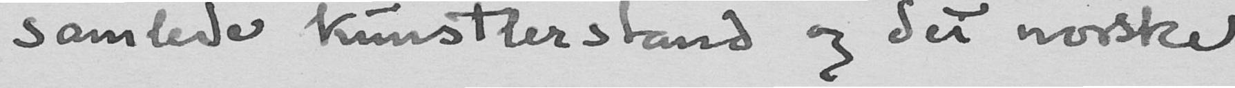samlede kunstlerstand og det norske
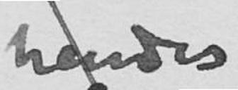hendes
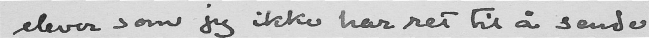elever som jeg ikke har ret til å sende
